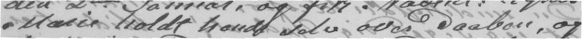Marie holdt hænde selv over Daaben, og
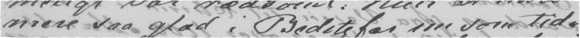mere saa glad i Bedstefar nu som tid-
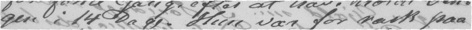gen i 14 Dage. Hun var for rask paa
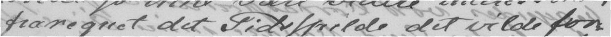fraregnet det Tidsspilde det vilde for-
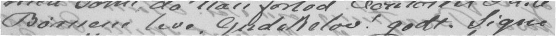Børnere leve Gudskelov! godt. Signe
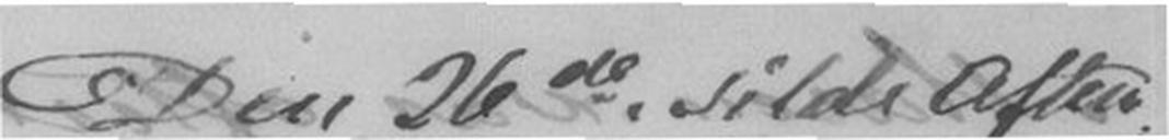Den 26de. silde Aften.
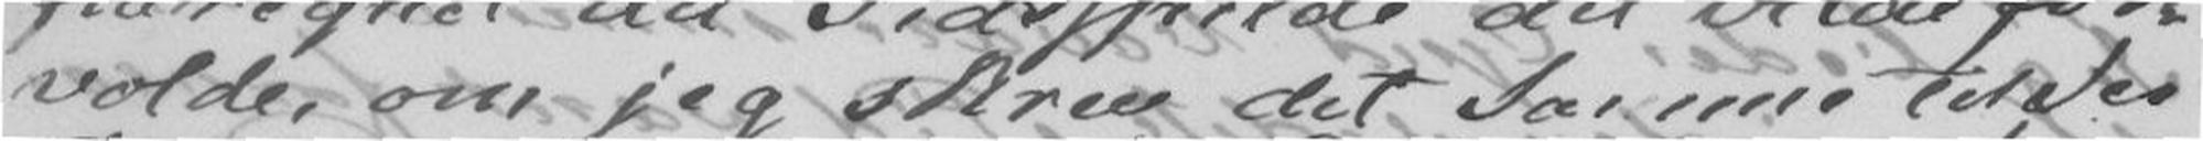volde, om jeg skrev det saa nu til Jer
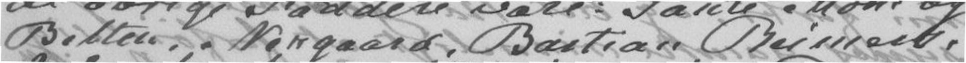Betten, Nergaard, Bastian Reimers,
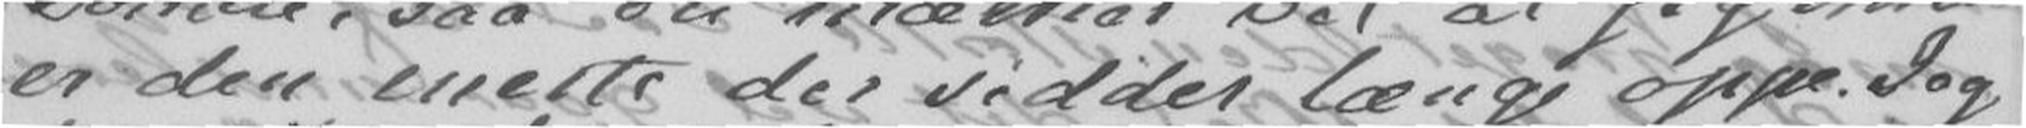er den eneste der sidder længe oppe. Jeg
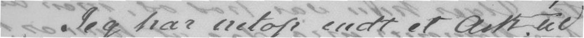Jeg har netop endt et Ark til
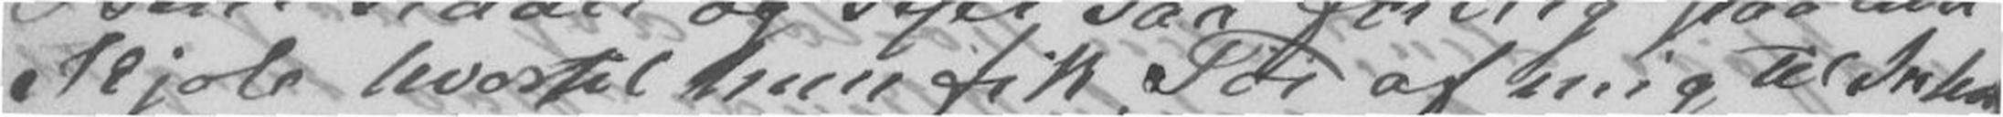Kjole hvortil hun fik Tøi af mig til Johns
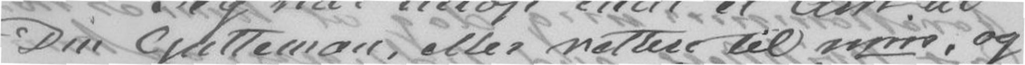Din Gutteman, eller rettere til min, og
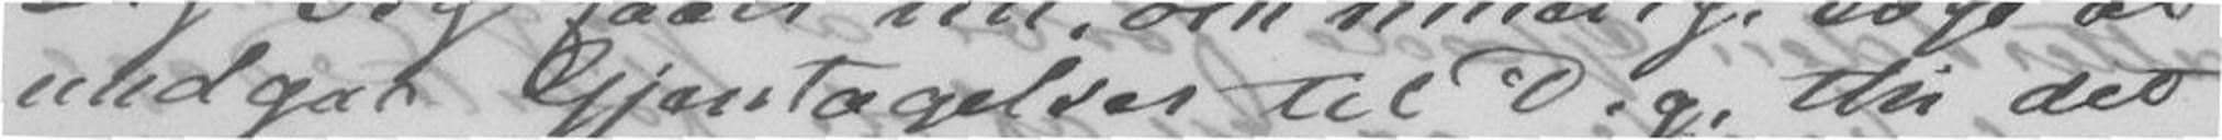undgaa Gjentagelser til Dig, thi det
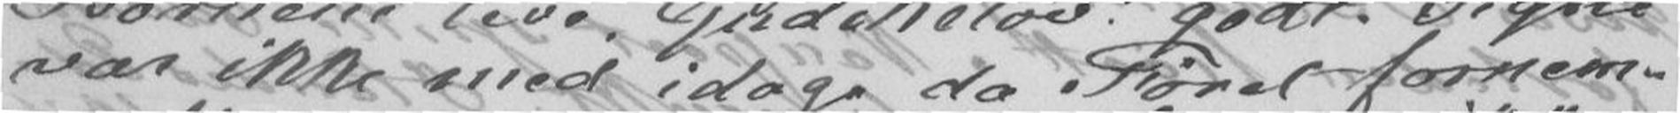var ikke med idag da Føret fornem-
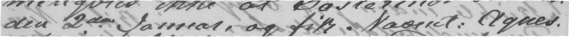den 2den Januar, og fik Navnet Agnes.
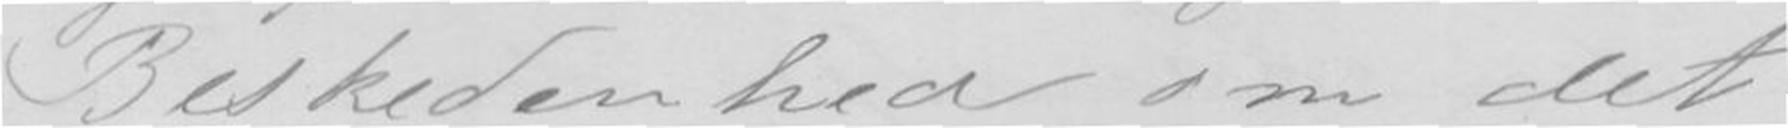Beskedenhed om det
